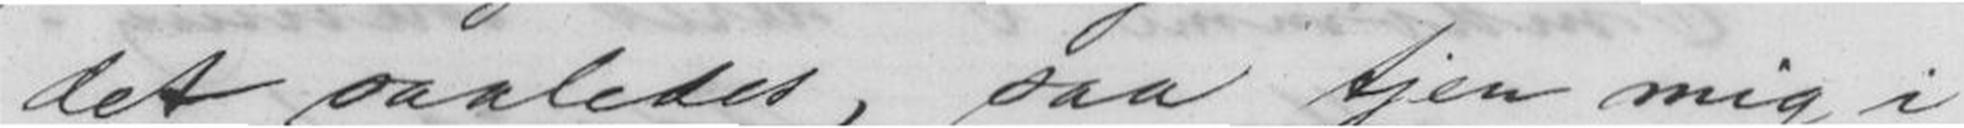det saaledes, saa tjen mig i
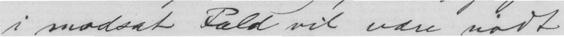i modsat Fald vil være nødt
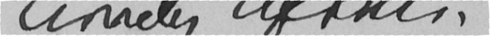Tirsdag Aften.
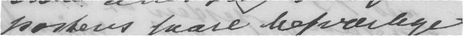posten saare besværlige
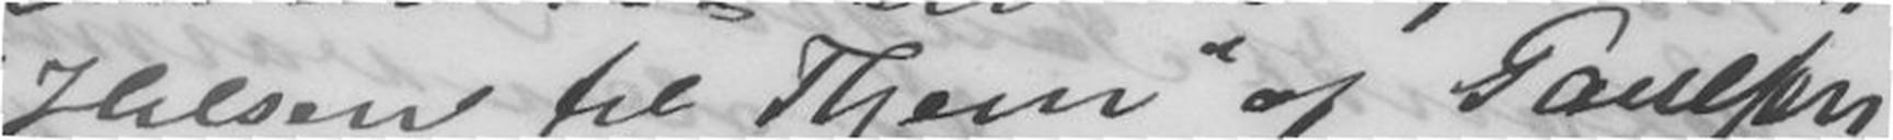"Hilsen til Thjem" af Paulsen
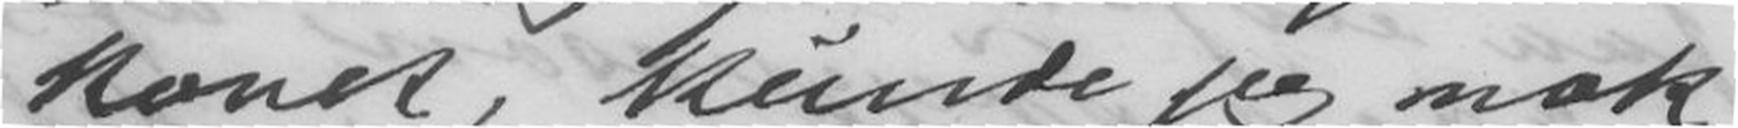koret, kunde jeg nok
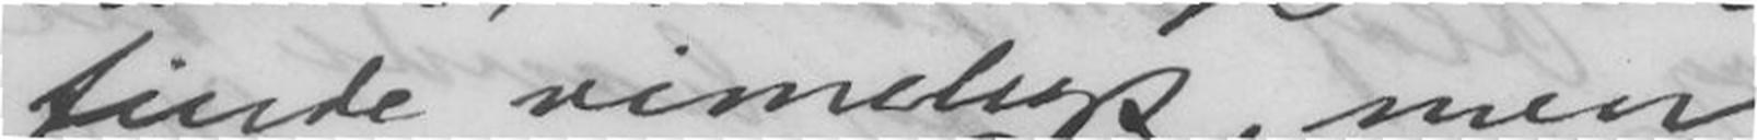finde rimeligt, men
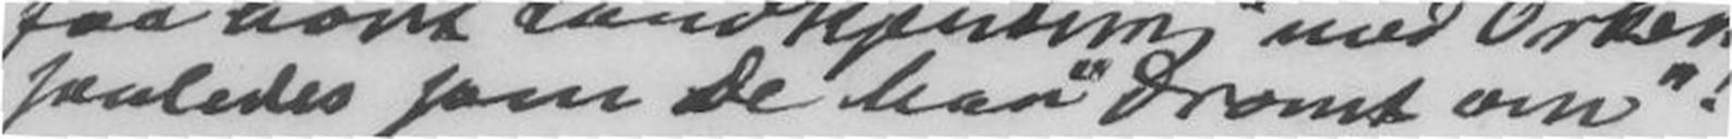saaledes som De har "Drømt om"!
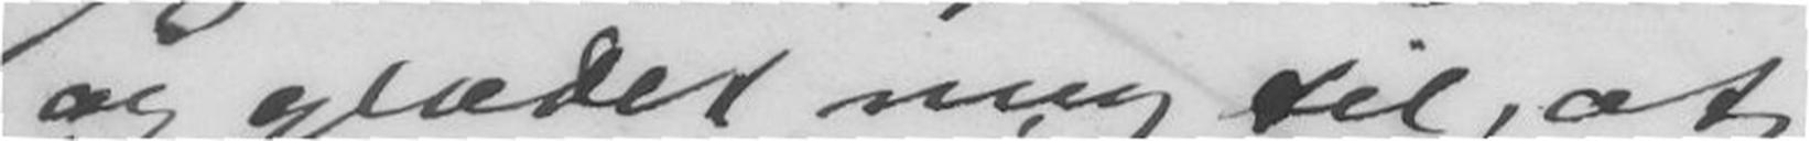og glædet mig til, at
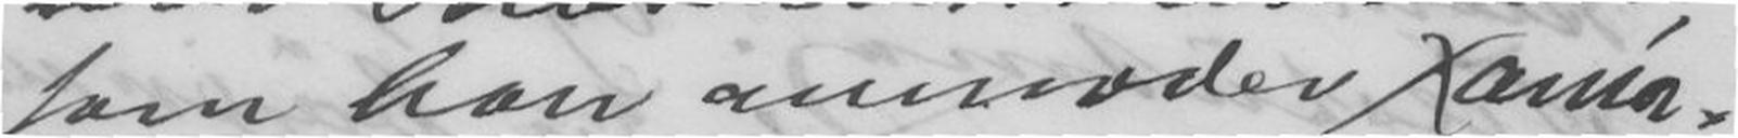som han anmoder Xania-
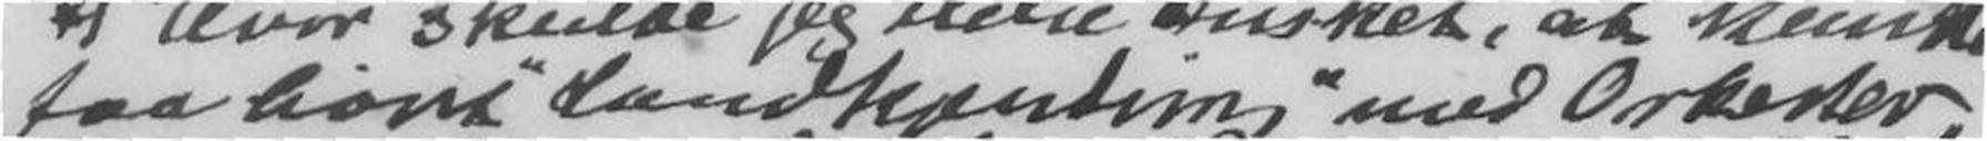faa hørt "Landkjænding" med Orkester,
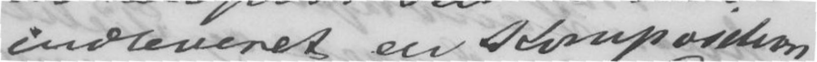indleveret en Komposition
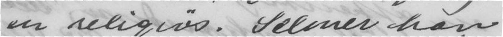en religiøs. Selmer har
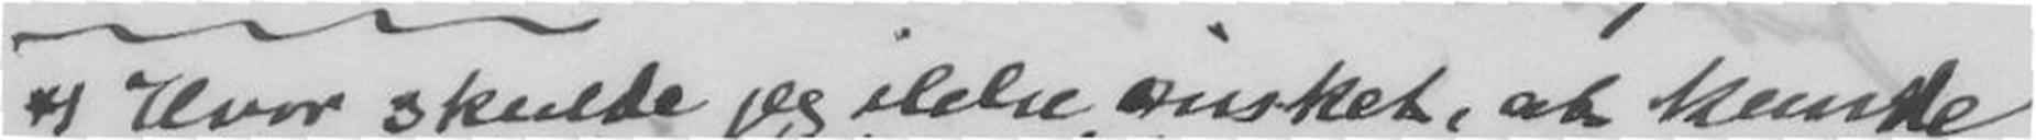*) Hvor skulde jeg ikke ønsket at kunde
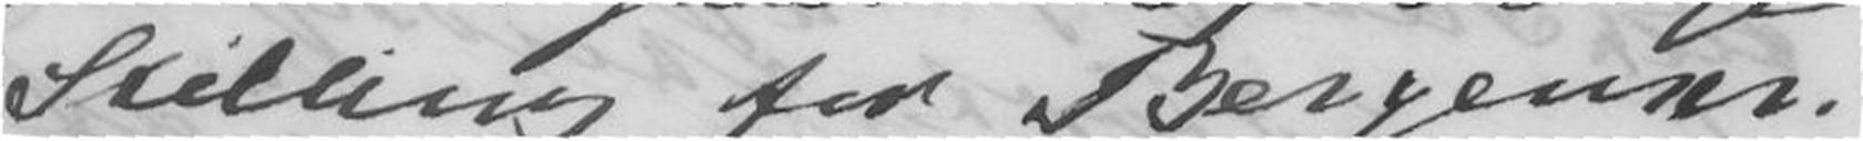Stilling for Bergenser-
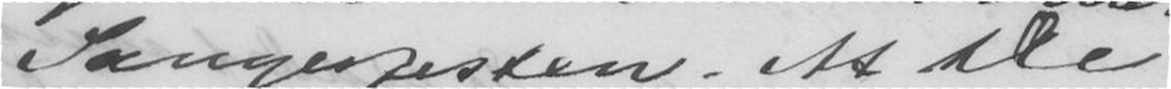Sangerfesten. At De
